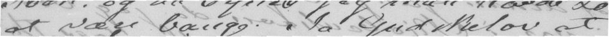at være bange. Ja Gudskelov at
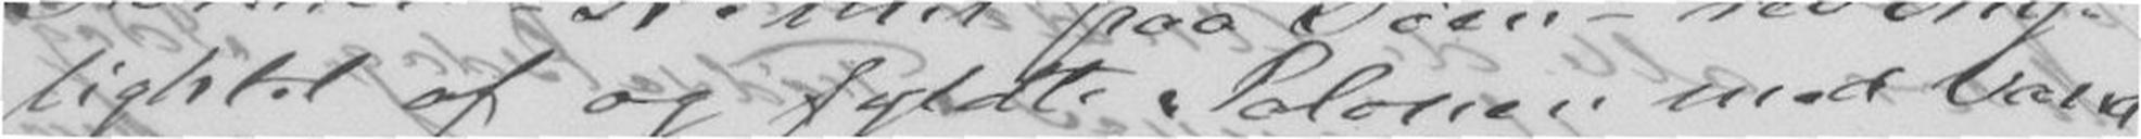lightet af og fyldte Salonen med Vand,
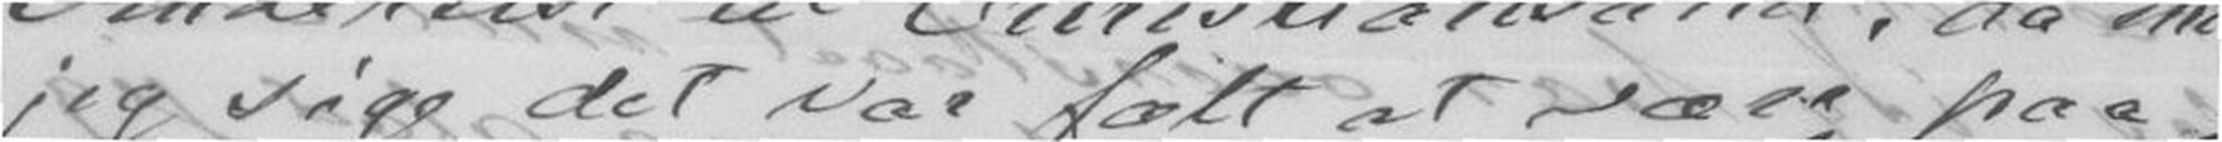jeg sige det var fælt at være paa
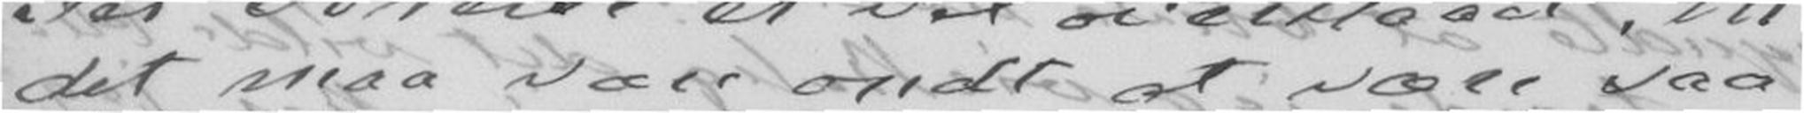det maa være ondt at være saa
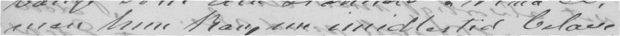men hun kan nu imidlertid belave
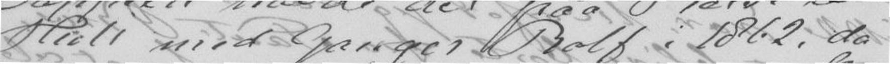Hull med Ganger Rolf i 1862, da
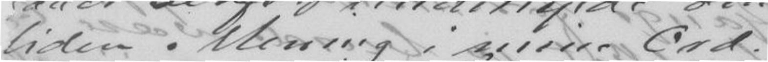liden Mening i mine Ord.
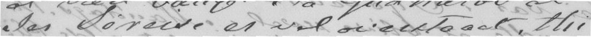Jer Søreise er vel overstaaet, thi
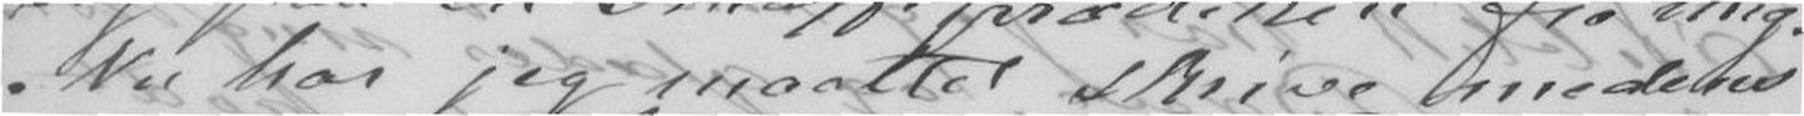Nu har jeg maattet skrive medens
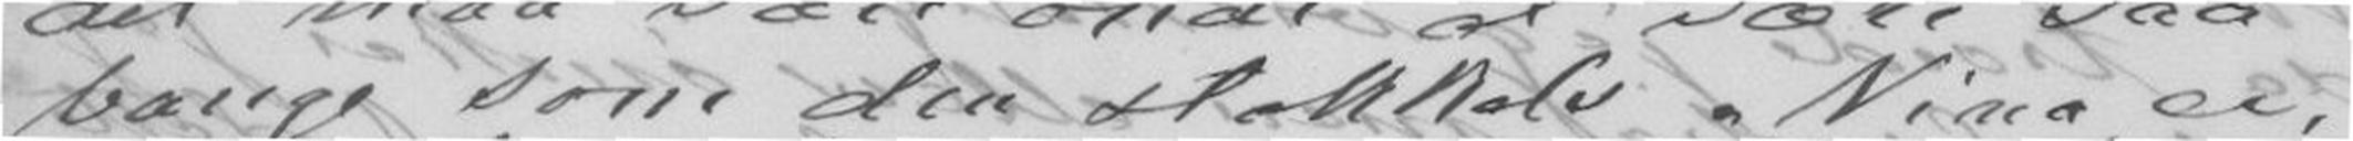bange som den stakkels Nina er,
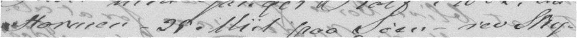Stormen - 25 Miil paa Søen - rev Sky-
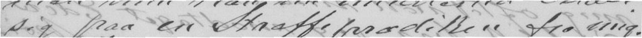sig paa en Straffeprædiken fra mig.
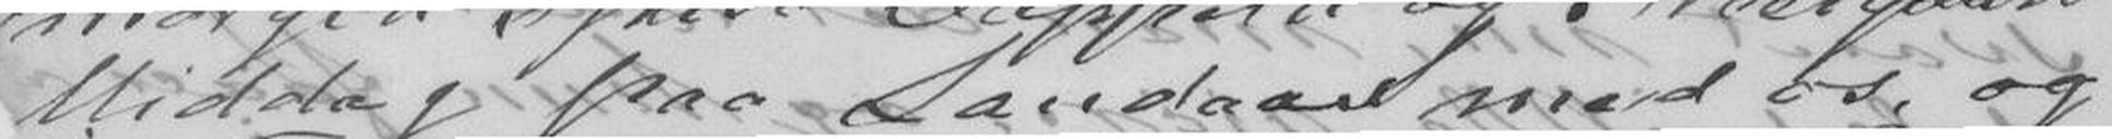Middag paa Landaas med os, og
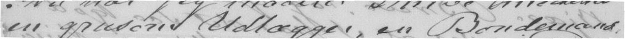en grusom Udlægger, en Bondemand
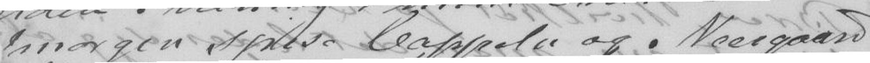morgen spiise Cappeln og Neergaard
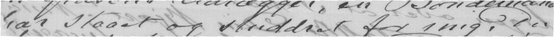har staaet og sluddret for mig, Du
